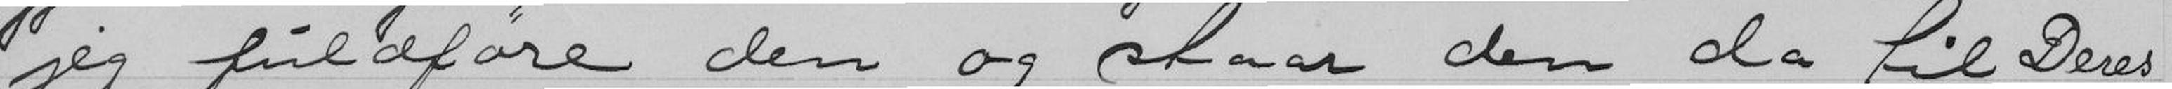jeg fuldføre den og staar den da til Deres
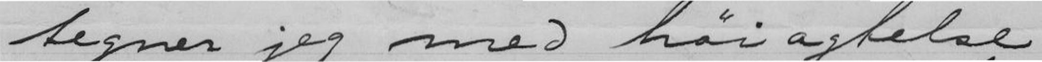tegner jeg med høiagtelse
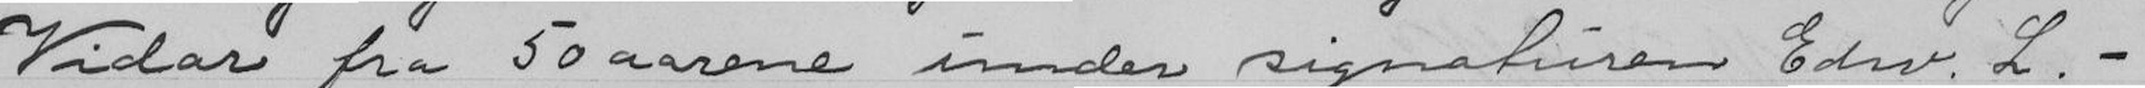Vidar fra 50 aarene under signaturen Edv.L.-
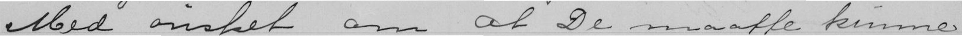Med ønsket om at De maatte kunne
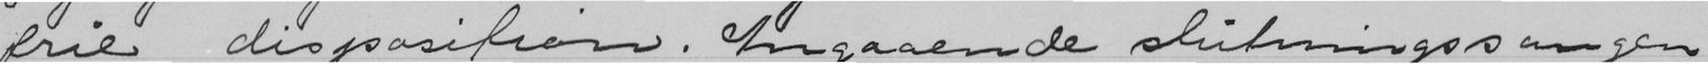frie disposition. Angaaende slutningssangen
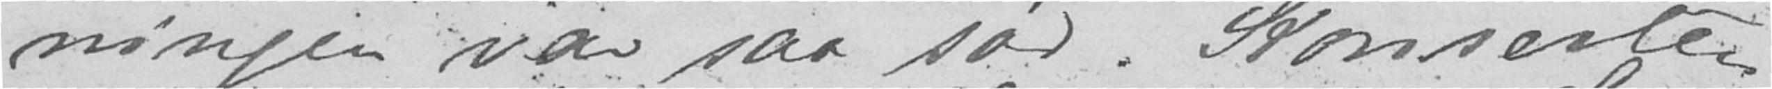ningen var saa sød. Konserten
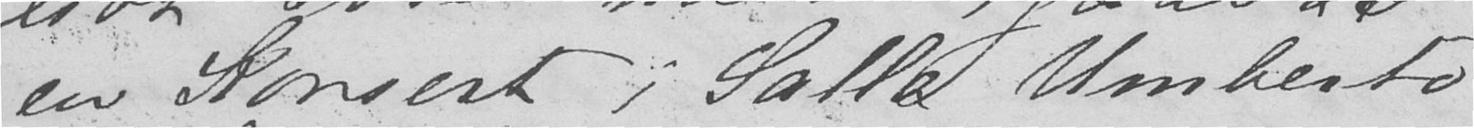en Konsert i Salle Umberto.
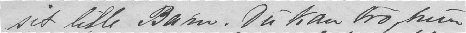sit lille Barn. Du kan tro, hun
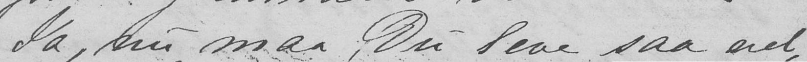Ja, nu maa Du leve saa vel,
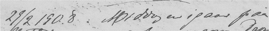29/2 1908. Middagen igaar paa
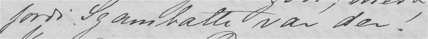fordi Sg ambatti var der!
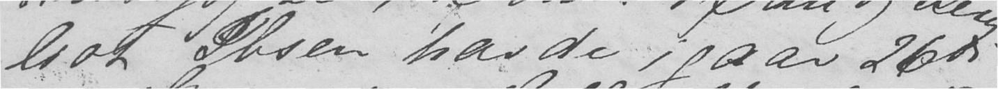liot Ibsen havde igaar 26de
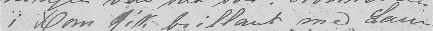i Rom gik brillant med Laur-
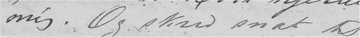mig. Og skriv snart til
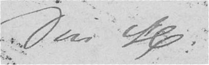Din H.
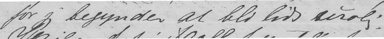for jeg begynder at bli lidt urolig.
